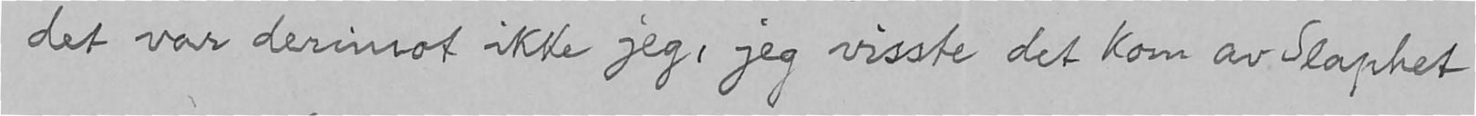det var derimot ikke jeg, jeg visste det kom av Slaphet
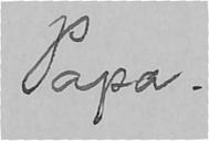Papa.
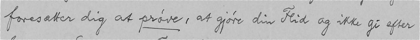foresætter dig at prøve, at gjøre din Flid og ikke gi efter
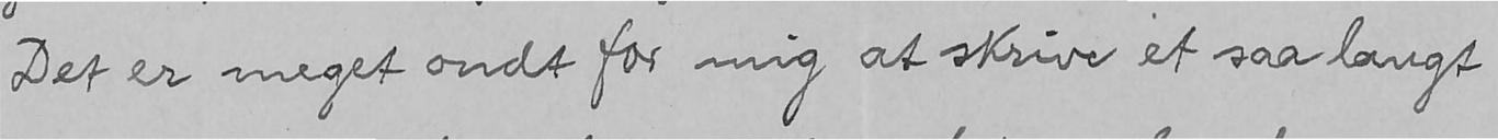Det er meget ondt for mig at skrive et saa langt
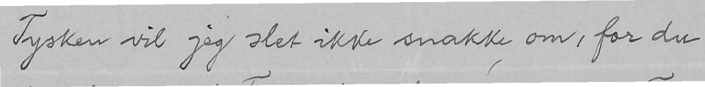Tysken vil jeg slet ikke snakke om, for du
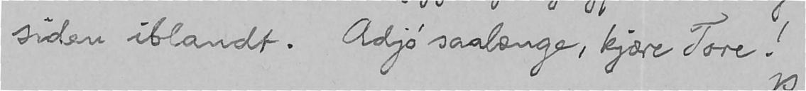siden iblandt. Adjø saalænge, kjære Tore!
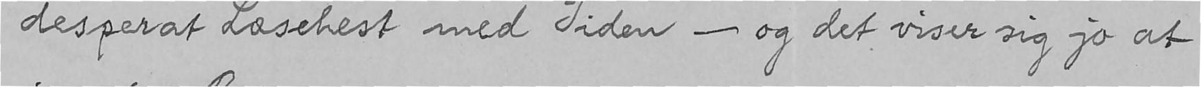desperat Læsehest med Tiden - og det viser sig jo at
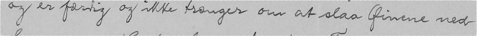og er færdig og ikke trænger om at slaa Øinene ned
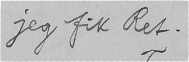jeg fik Ret.
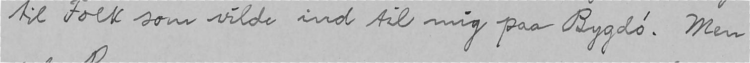til Folk som vilde ind til mig paa Bygdø. Men
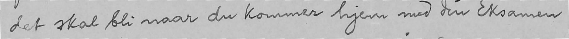det skal bli naar du kommer hjem med din Eksamen
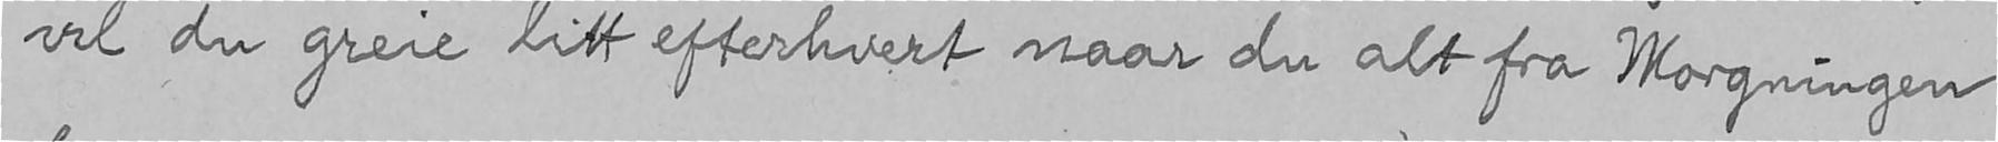vil du greie litt efterhvert naar du alt fra Morgningen
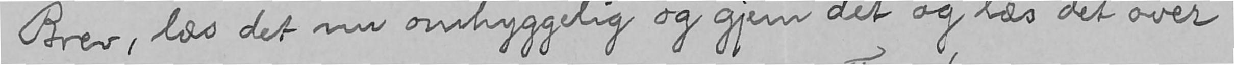Brev, læs det nu omhyggelig og gjem det og læs det over
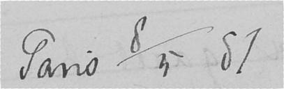Paris 8/5 87
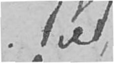ved
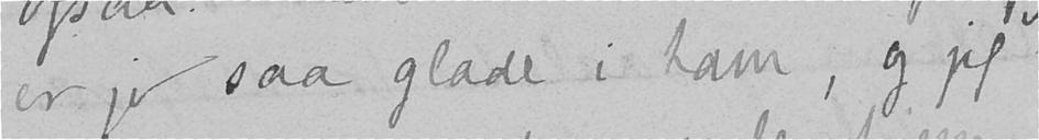er jo saa glade i ham, og jeg
